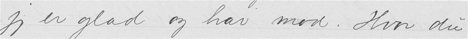jeg er glad og har mod. Hvor du
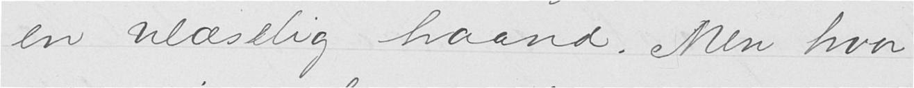en ulæselig haand. Men hvor
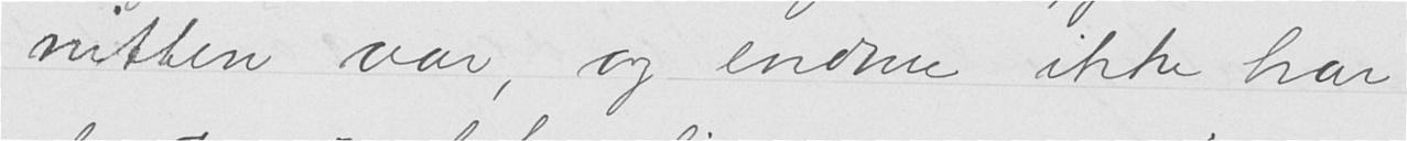nitten aar, og endnu ikke har
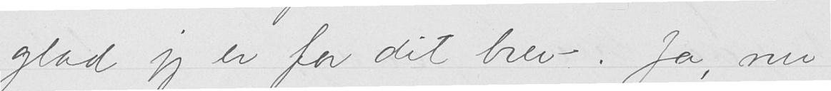glad jeg er for dit brev. Ja, nu
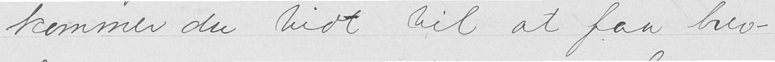kommer du tidt til at faa brev
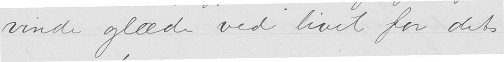vinde glæde ved livet for dets
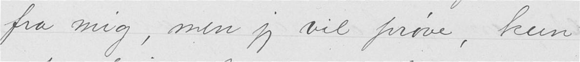fra mig, men jeg vil prøve, kun
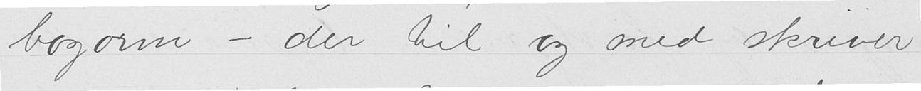bogorm - der til og med skriver
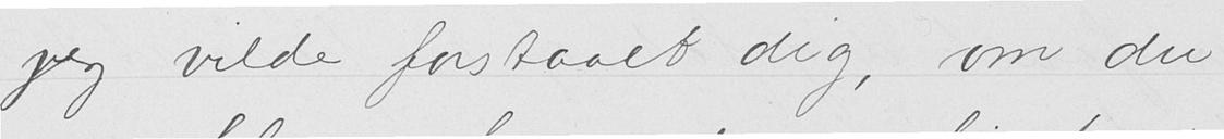jeg vilde forstaaet dig, om du
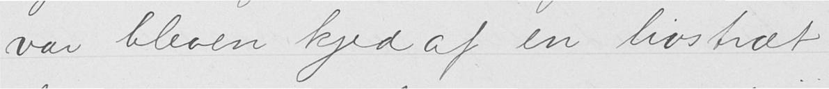var bleven kjed af en livstræt
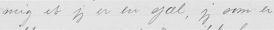mig at jeg er en sjæl, jeg som er
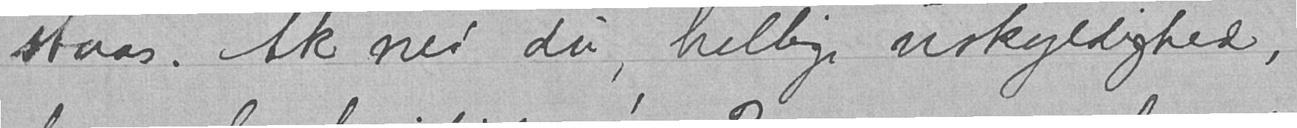staas. Ak nei du, hellige uskyldighed,
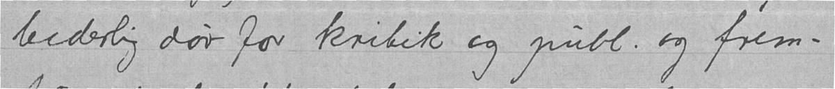bederlig døv for kritik og publ. og frem-
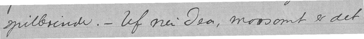spillerinde. - Uf nei Dea, morsomt er det
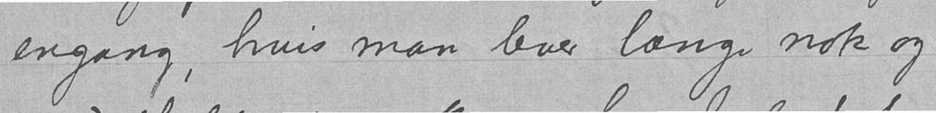engang, hvis man lever længe nok og
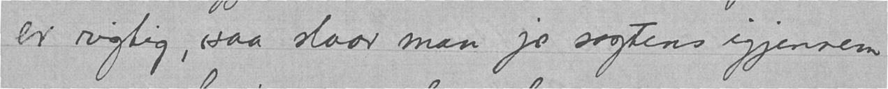er rigtig, saa slaar man jo sagtens igjennem
